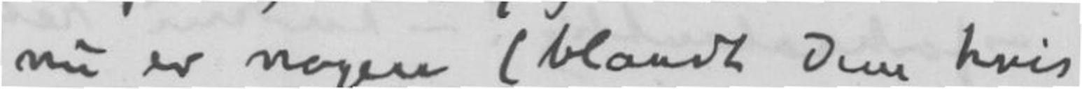nu er nogen (blandt dem hvis
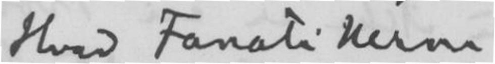Hvad Fanatikerne
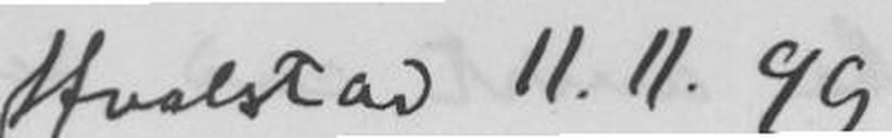Hvalstad 11.11.99
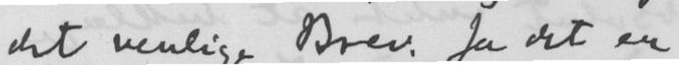det venlige Brev. Ja det er
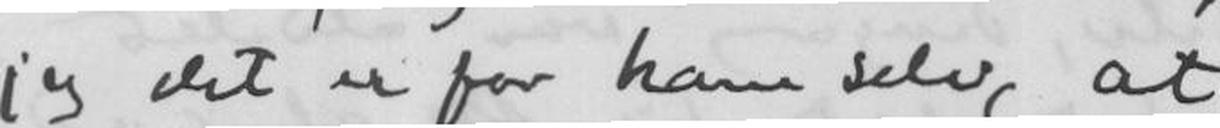jeg det er for ham selv, at
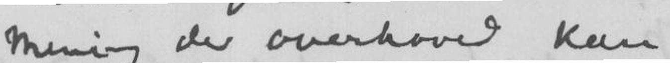Mening der overhoved kan
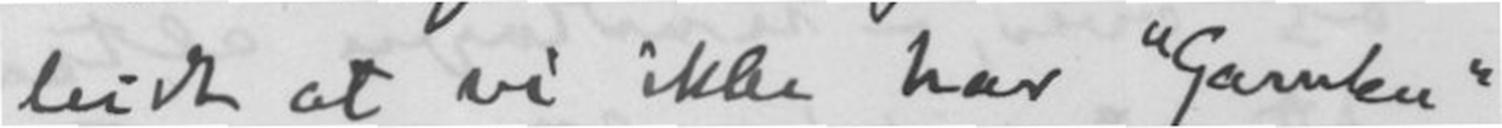leidt at vi ikke har "Gamlen"
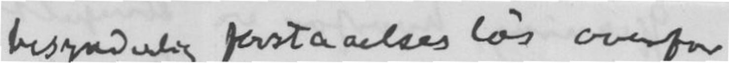besynderlig forstaaelsesløs overfor
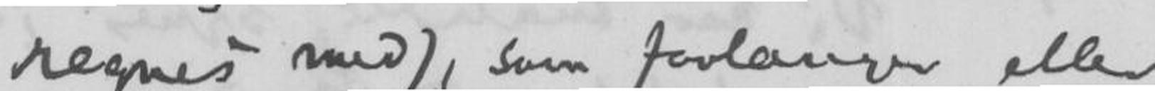regnes med), som forlanger eller
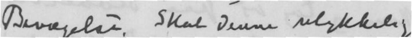Bevægelse. Skal denne ulykkelige
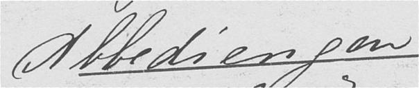Abbediengen
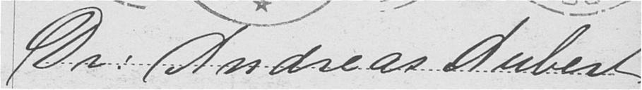Dr. Andreas Aubert
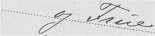og Frue
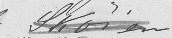Skøien
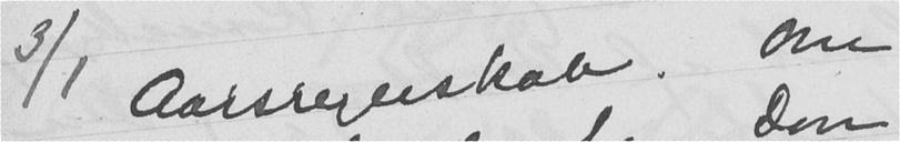3/1 Aarsregnskab. Om
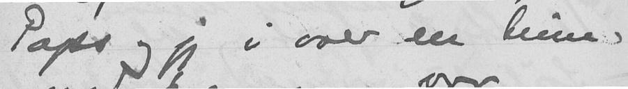Paps og jeg i over en time,
